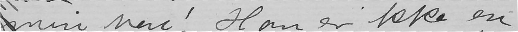min ven! Han er ikke en
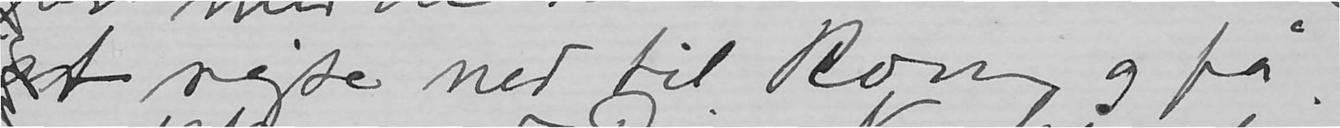at rejse ned til Rom og få
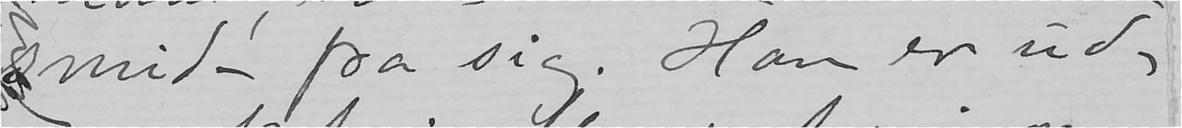smide fra sig. Han er ud-
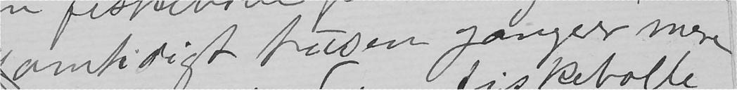samtidigt tusen ganger mere
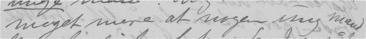meget mere at nogen ung mand
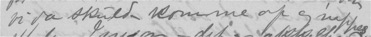vi da skulde komme op og nappes.
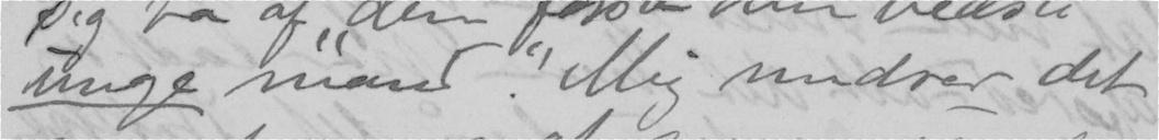unge mand". Mig undrer det
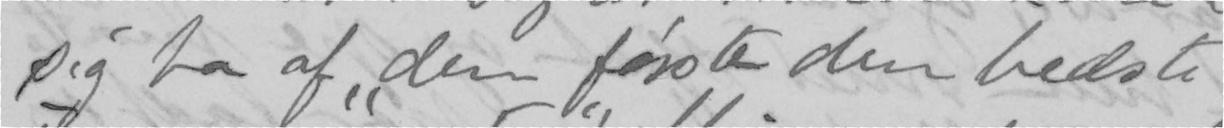sig ta af, "den første den bedste
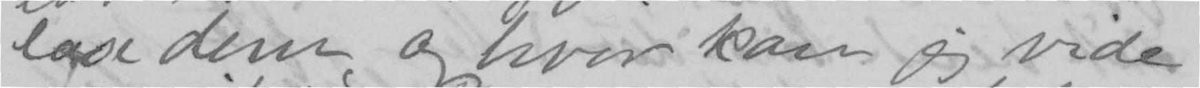læse dem og hvor kan jeg vide
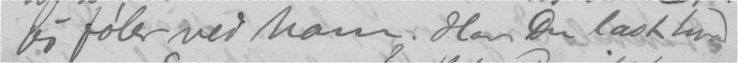jeg føler ved ham. Har Du læst hvad
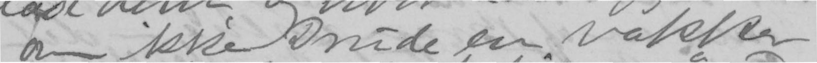om ikke Drude en vakker
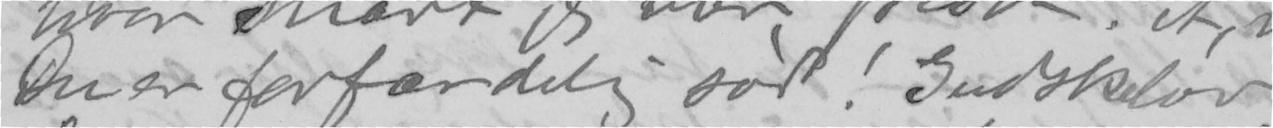Du er forfærdelig sød! Gudskelov
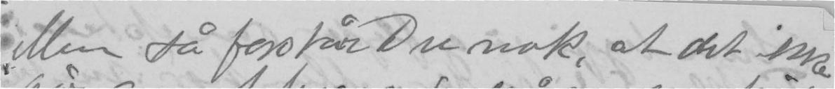Men så forstår Du nok at det ikke
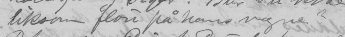liksom flou på hans vegne?
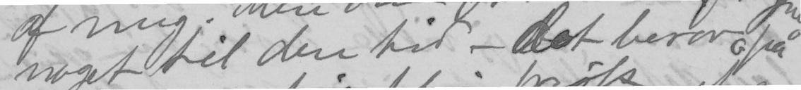noget til den til - det beror på
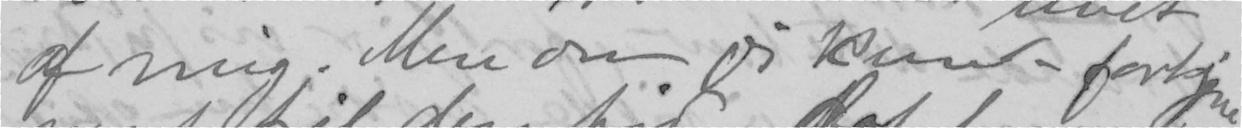af mig. Men om jeg kunde fortjene
